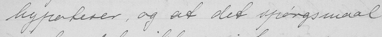hypoteser, og at det spørgsmaal
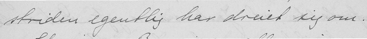striden egentlig har dreiet sig om.
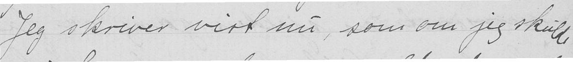Jeg skriver vist nu, som om jeg skulle
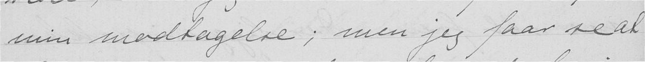min modtagelse; men jeg faar se at
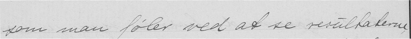som man føler ved at se resultaterne
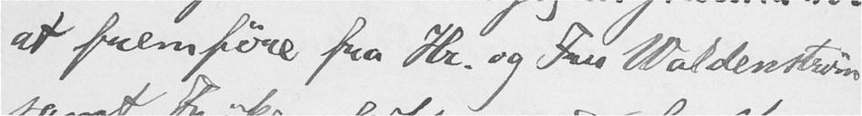at fremføre fra Hr. og Fru Waldenstrøm
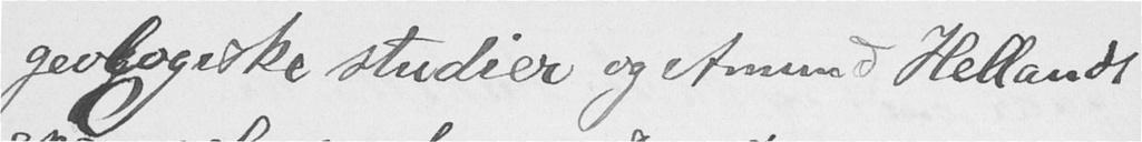geologiske studier og Amund Hellands
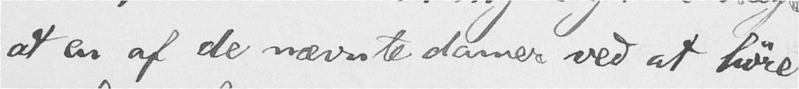at en af de nævnte damer ved at høre
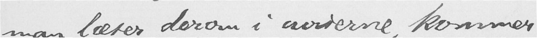man læser derom i aviserne, kommer
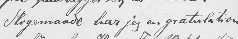I ligemaade har jeg en gratulation
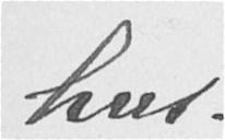hus.
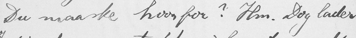Du maaske hvorfor? Hm. Dog lader
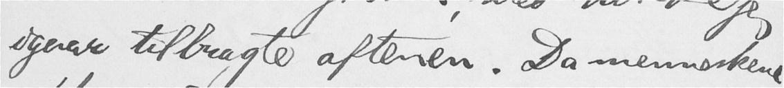igaar tilbragte aftenen. Da menneskene
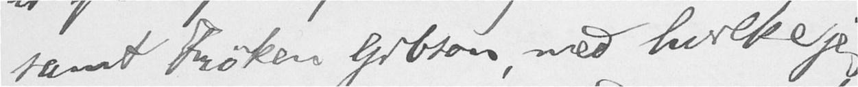samt frøken Gibson, med hvilke jeg
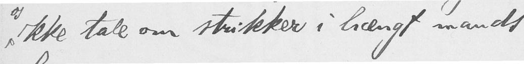ikke tale om strikker i hængt mands
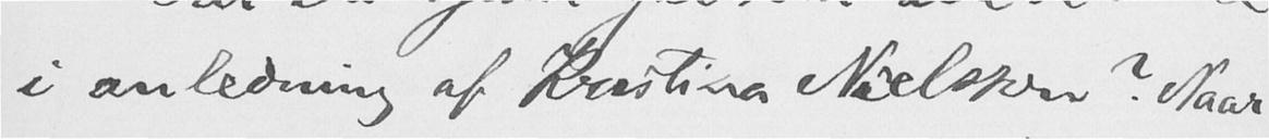i anledning af Kristina Nielsson? Naar
